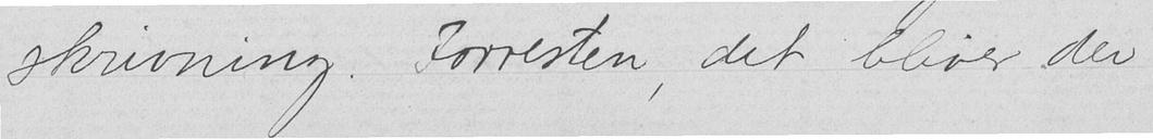skrivning. Forresten, det bliver der
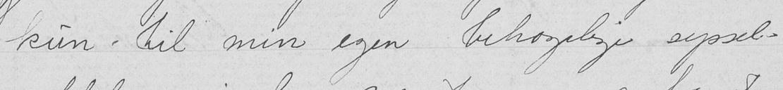kun til min egen behagelige syssel-
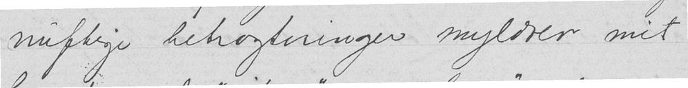nuftige betragtninger myldrer mit
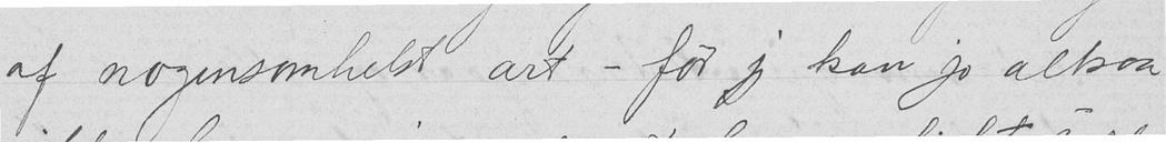af nogensomhelst art - for jeg kan jo altsaa
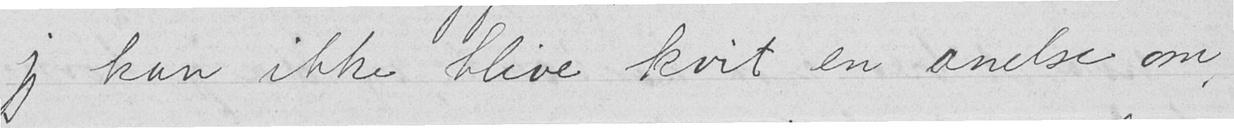jeg kan ikke blive kvit en anelse om,
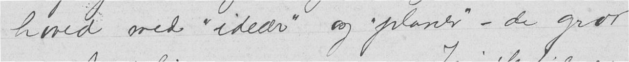hoved med "ideer" og "planer" - de gror
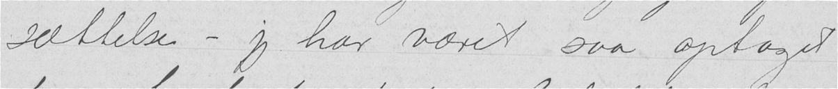sættelse - jeg har været saa optaget
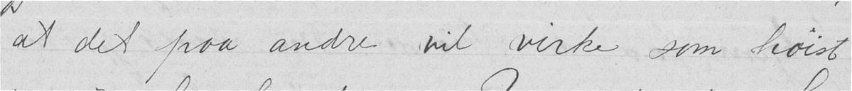at det paa andre vil virke som høist
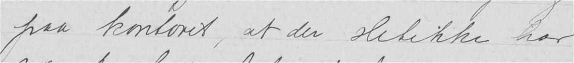paa kontoret, at der sletikke har
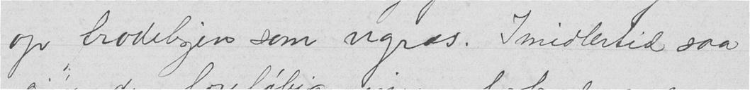op frodeligen som ugræs. Imidlertid saa
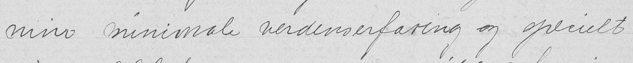min minimale verdenserfaring og specielt
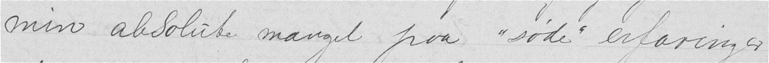min absolute mangel paa "søde" erfaringer
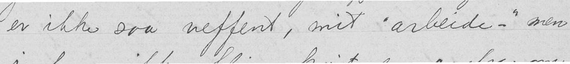er ikke saa ueffent, mit "arbeide" - men
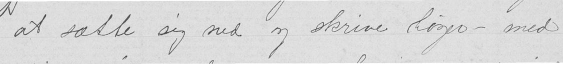at sætte sig ned og skrive bøger - med
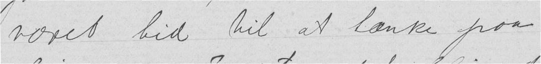været tid til at tænke paa
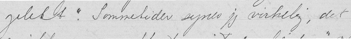gelebt". Sommetider synes jeg virkelig, det
